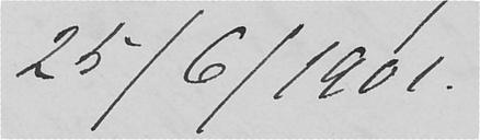25/6/1901.
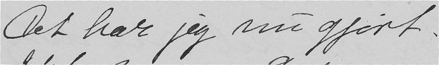Det har jeg nu gjort.
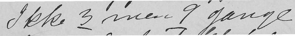Ikke 3 men 9 gange
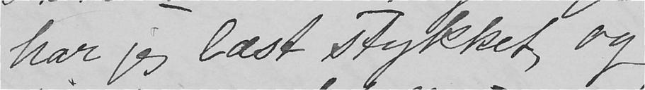har jeg læst stykket, og
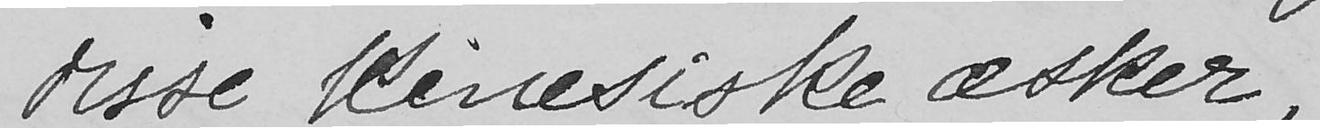disse kinesiske æsker,
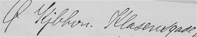Ø Kjbhvn. Klasensgade
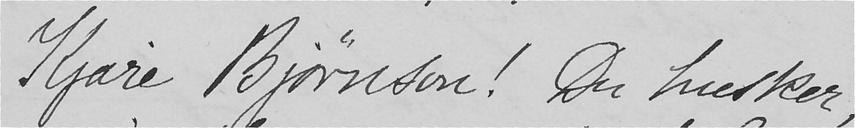Kjære Bjørnson! Du husker,
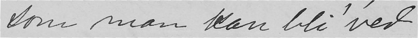som man kan bli ved
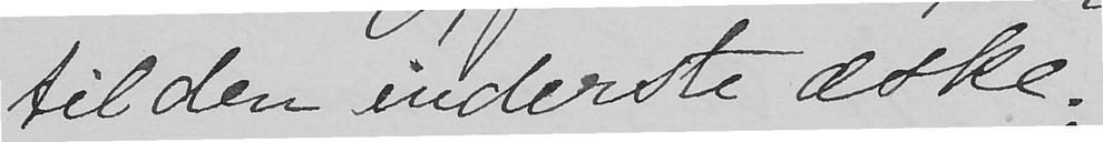til den inderste æske.
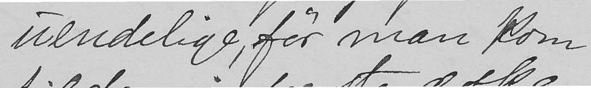uendelige, før man kom
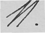11.
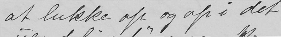at lukke op og op i det
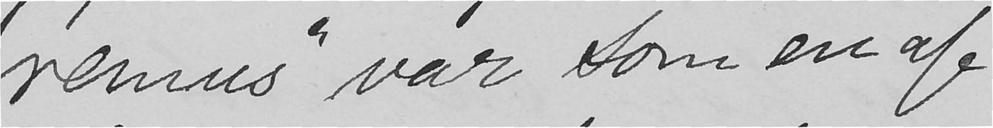remus" var som en af
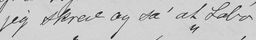jeg skrev og sa' at "Labo
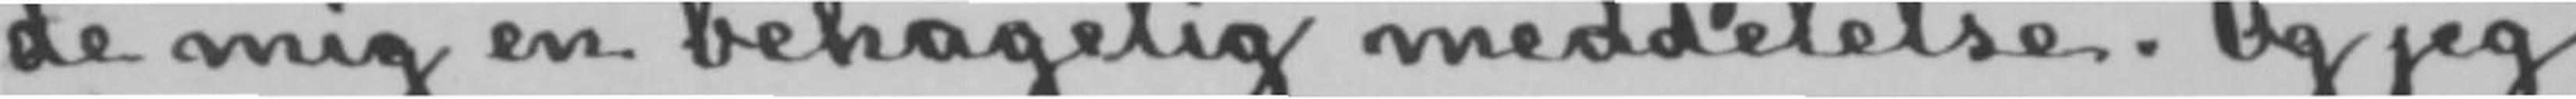de mig en behagelig meddelelse. Og jeg
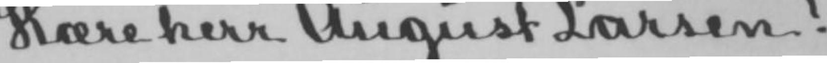Kære herr August Larsen!
